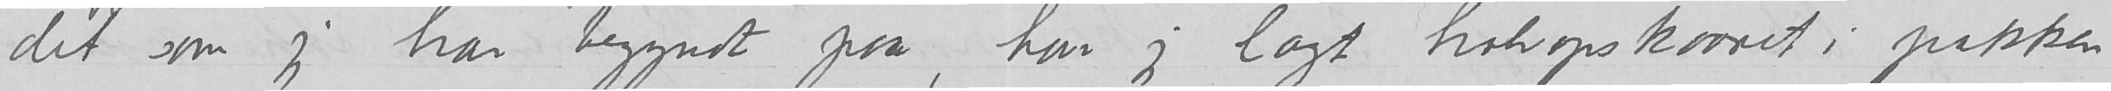det som jeg har begyndt paa, har jeg lagt halvopskaaret i pakken
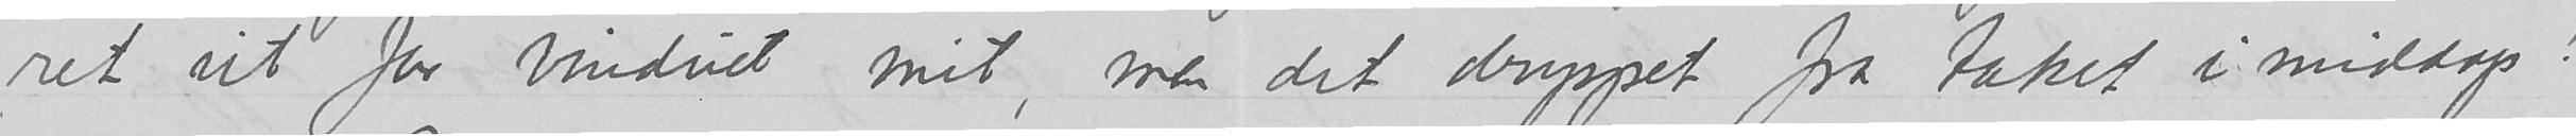ret ut for vinduet mit, men det dryppet fra taket i middags
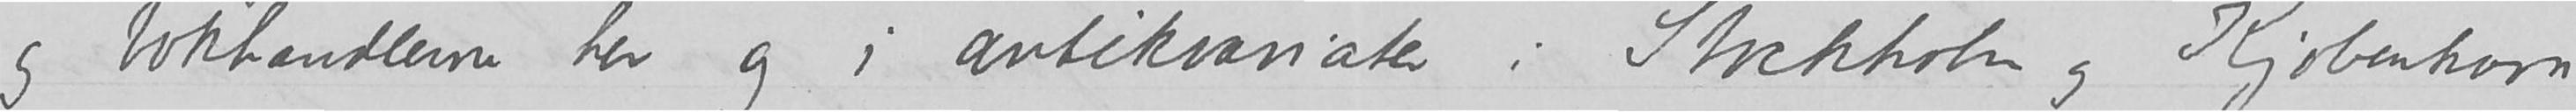og bokhandlerne her og i antikvariater i Stockholm og Kjøbenhavn
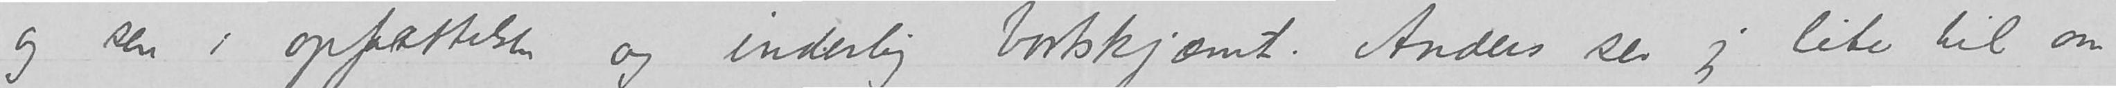og sen i oppfattelsen og inderlig bortskjæemt. Anders ser jeg lite til om
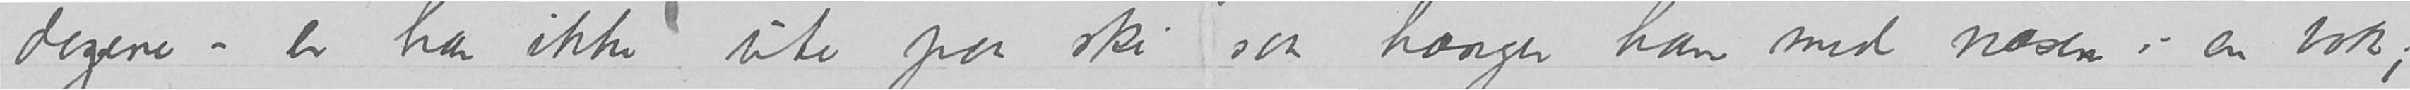dagene – er han ikke ute paa ski saa hænger han med næsen i en bok;
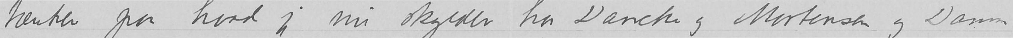tæenker paa hvad jeg nu skylder hos Dancke og Mortensen og Damm
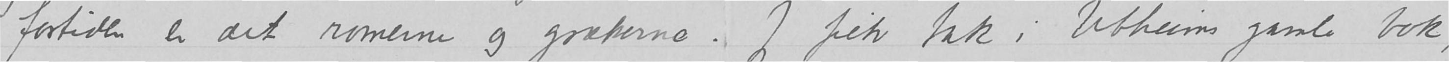fortiden er det romerne og grækerne.  Jeg fik tak i Utheims gamle bok,
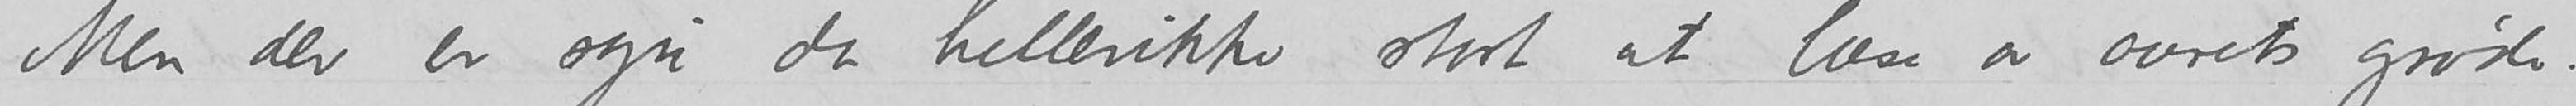Men der er også da hellerikke stort at læse av aarets grøde.
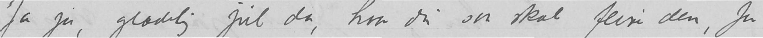Ja ja, glædelig jul da, hvor du saa skal feire den, for
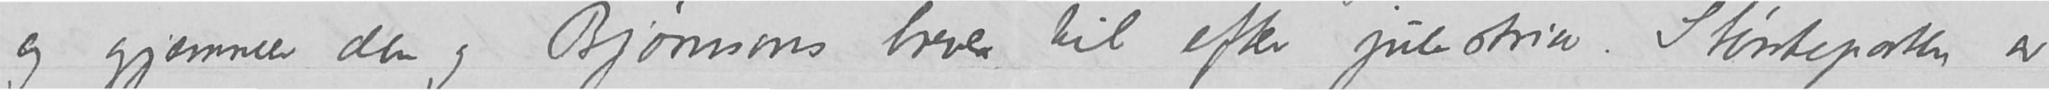anbefaling og gjæemmer den og Bjørnsons brever til efter julestria. Størsteparten av
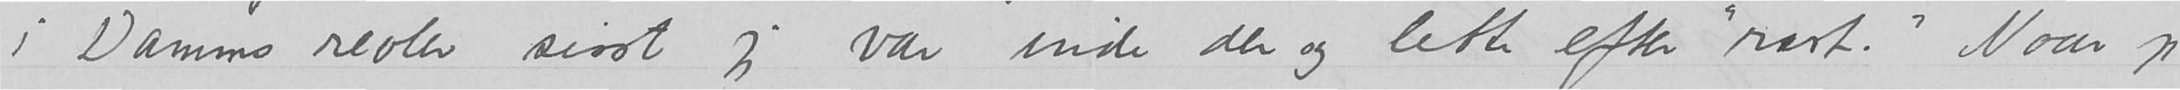i Damms reoler sisst jeg var inde der og lette efter «rart.». Naar jeg
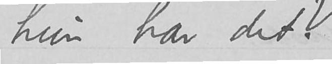hun har det?.
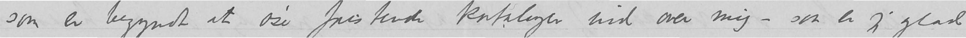som er begyndt at øse fristende kataloger ind over mig – saa er jeg glad
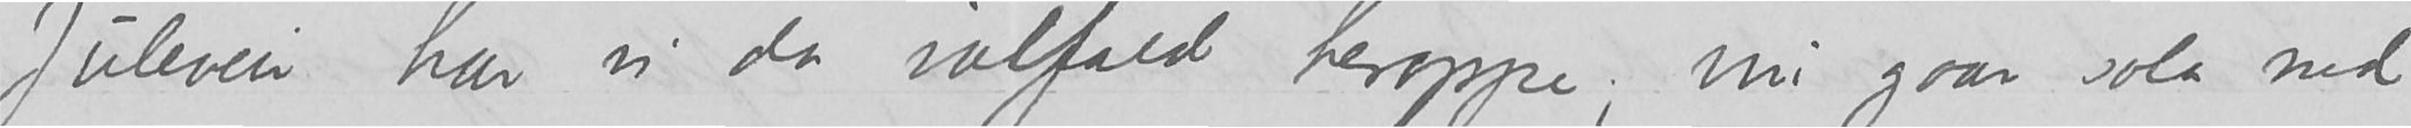Juleveir har vi da ialfald heroppe, nu gaar sola ned
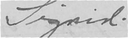Sigrid
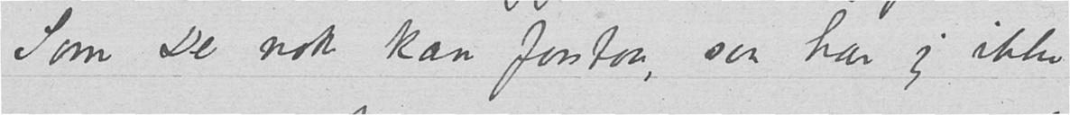Som De nok kan forstaa, saa har jeg ikke
